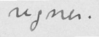regner.
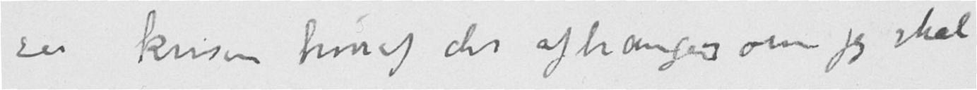saa krisen hvoraf det afhænger om jeg skal
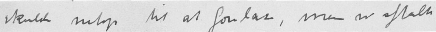skulde netop til at forelæse, men vi aftalte
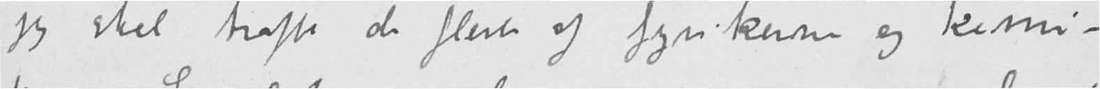jeg skal træffe de fleste af fysikerne og kemi-
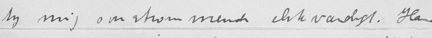tog mig overstrømmende elskværdigt. Han
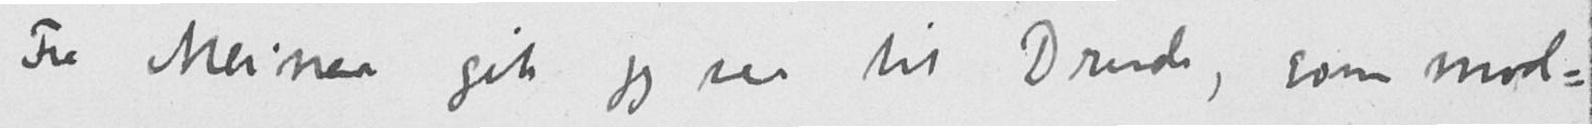Fra Meiner gik jeg saa til Drude, som mod-
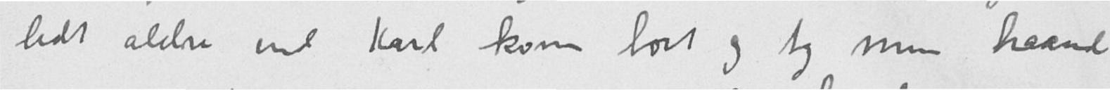lidt ældre end Karl kom bort og tog min haand
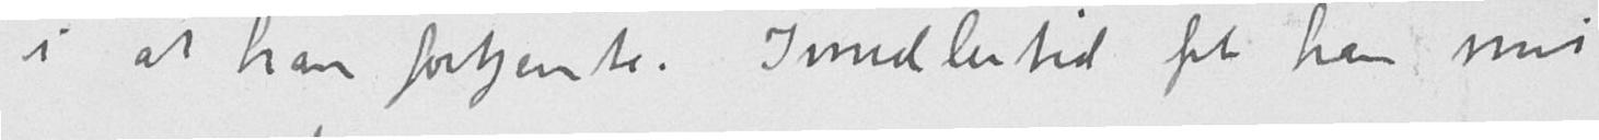i at han fortjente. Imidlertid fik han mit
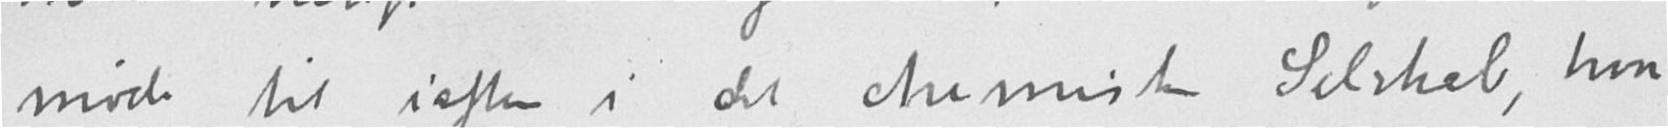møde til i aften i det chemiske Selskab, hvor
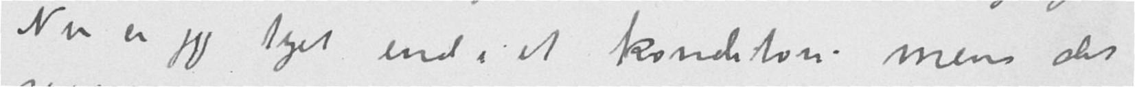Nu er jeg lyet ind i et konditori mens det
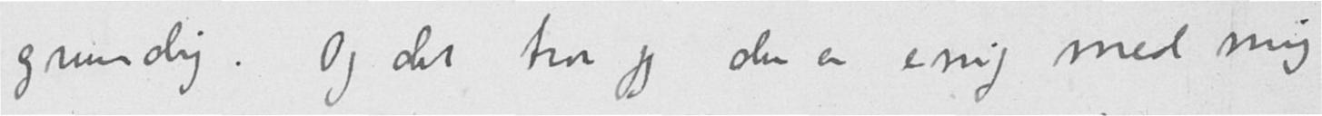grundig. Og det tror jeg du er enig med mig
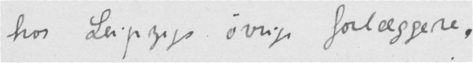hos Leipzigs øvrige forlæggere.
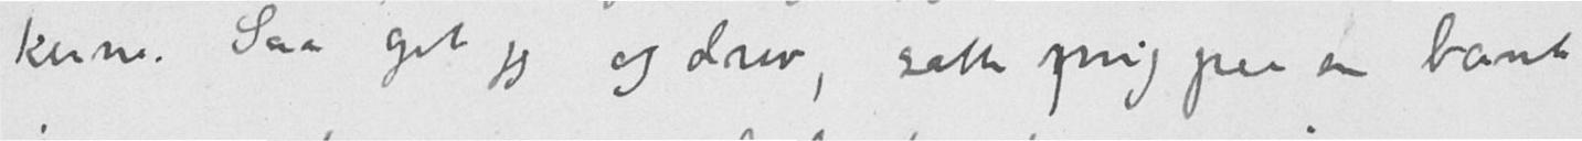kerne. Saa gik jeg og drev, sætte mig paa en bænk
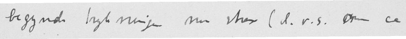begynde trykningen nu strax (d.v.s. om ca
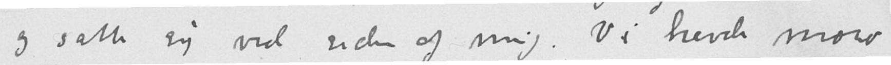og satte sig ved siden af mig. Vi havde moro
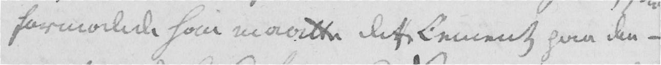formodede han maatte det Cement paa den-
